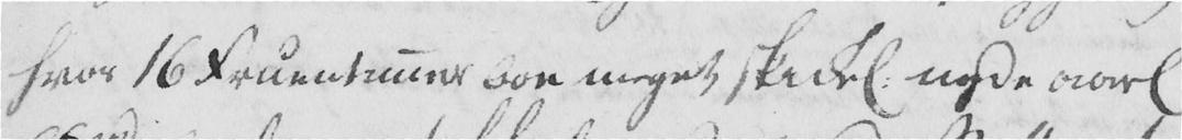hvor 16 Fruentimer boe meget skickel. nyde aarl
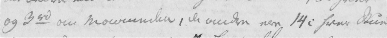og 3 rd om Maaneden, de andre ere 14 i hver Stue
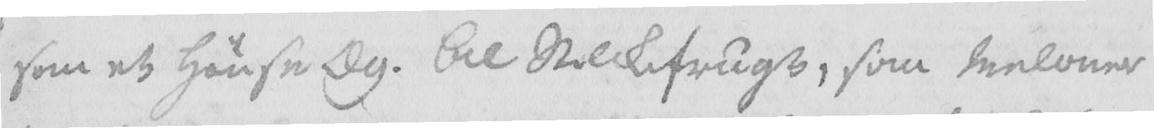som et Hønse Æg. Al Stilckefrugt, som Meloner
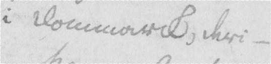i Danmarck, deri-
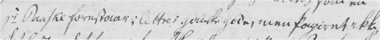Hr Paaske forestaar; lettres ganske gode, men Papiret ikke
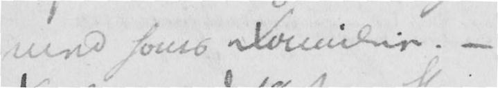med hans Familie. -
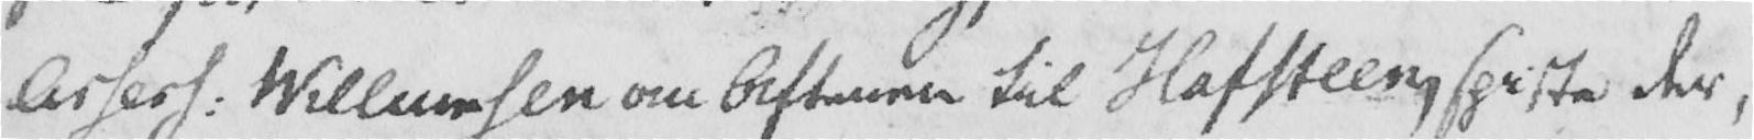Assess. Willumsen om Aftenen til Hafsteen, spiste der,
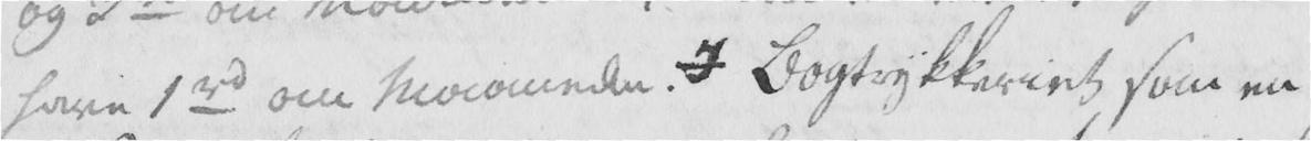have 1 rd om Maaneden. I Bogtrykkeriet som en
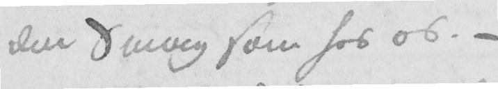den Smag som hos os. -
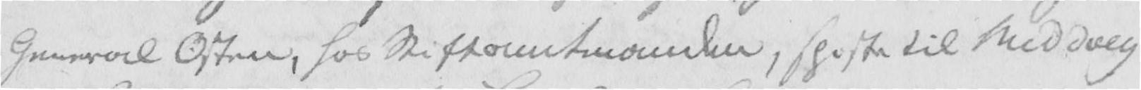General Osten, hos Stiftamtmanden, spiste til Middag
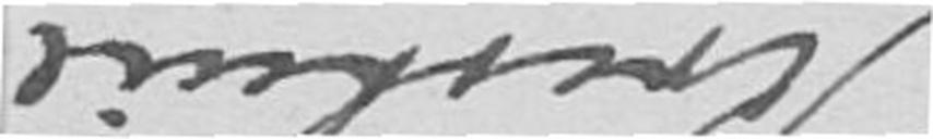Kristine
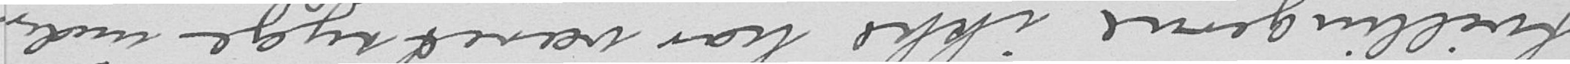tvillingerne ikke har været syge under
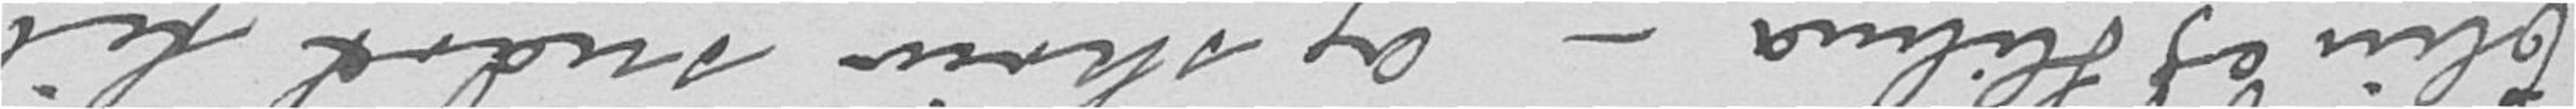Elin og Hilma - og skriv snart til
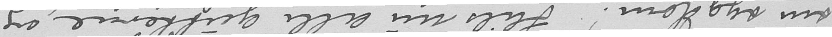sin sygdom! Hils nu alle gutterne, og
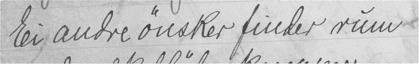Ei andre ønsker finder rum
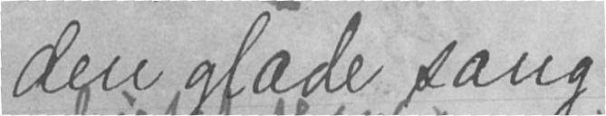den glade sang
